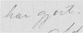har gjort.
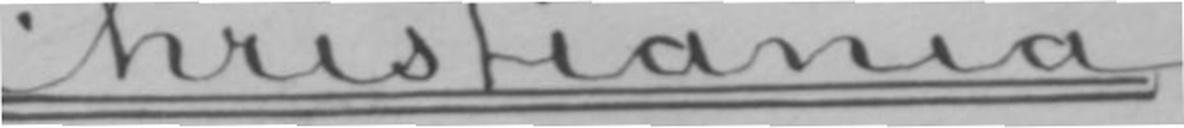Christiania.
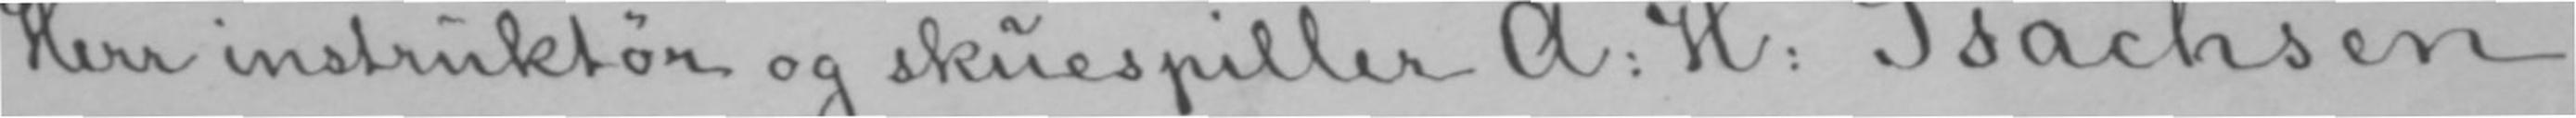Herr instruktør og skuespiller A: H: Isachsen.
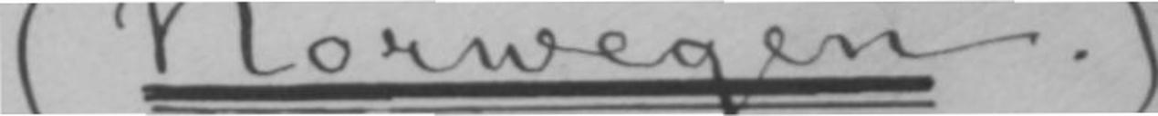(Norwegen.)
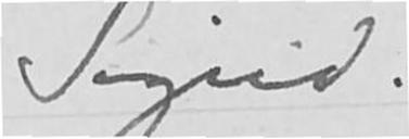Sigrid.
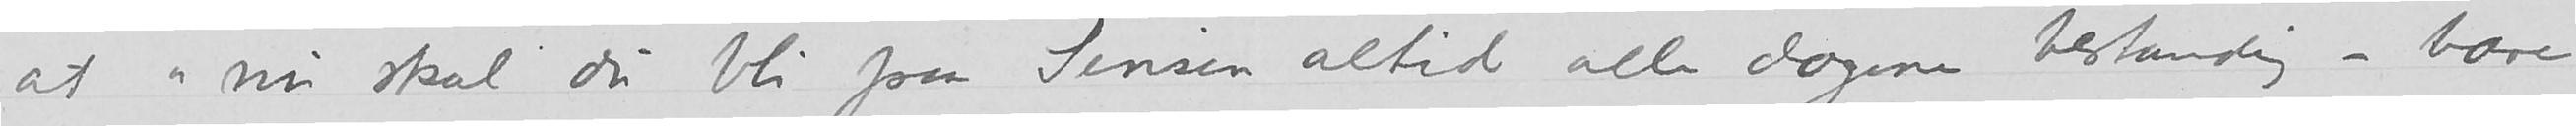at «nu skal du bli paa Sinsen altid alle dagene bestandig – bare
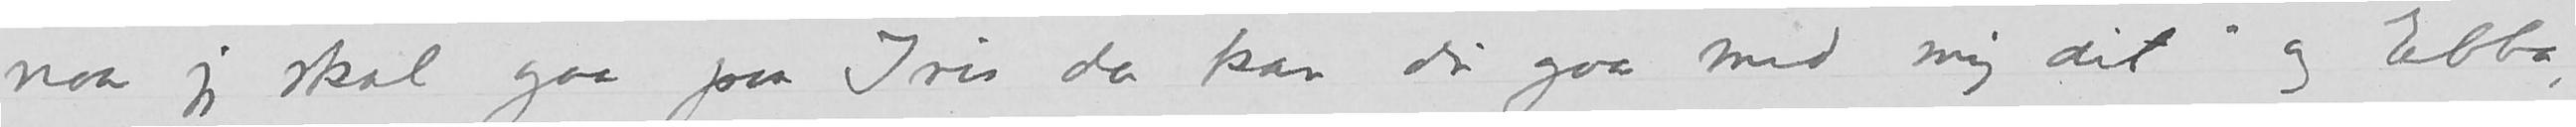naar jeg skal gaa paa Iris da kan du gaa med mig dit» og Ebba,
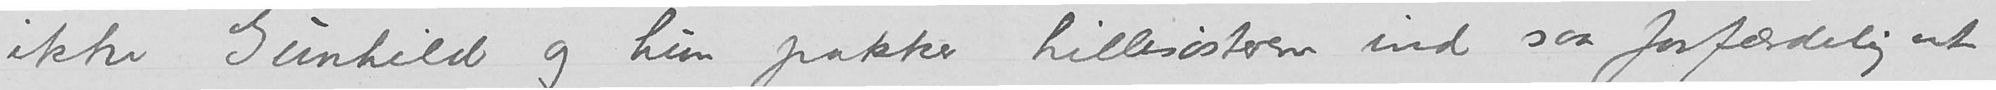ikke Gunhild og hun pakker Lillesøsteren ind saa forfærdelig at
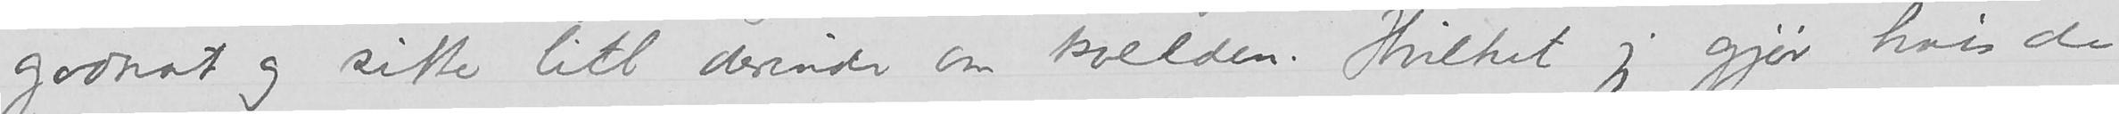godnat og sitte litt derinde om kvelden. Hvilket jeg gjør hvis de
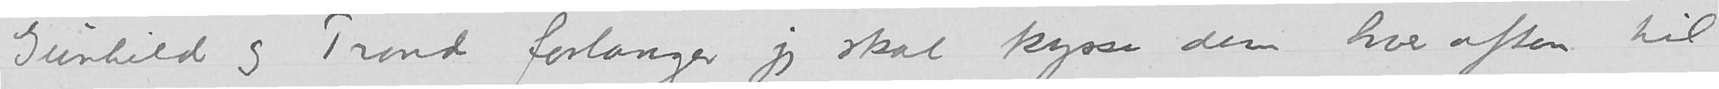Gunhild og Trond forlanger jeg skal kysse dem hver aften til
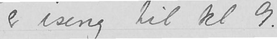er iseng til kl 9.
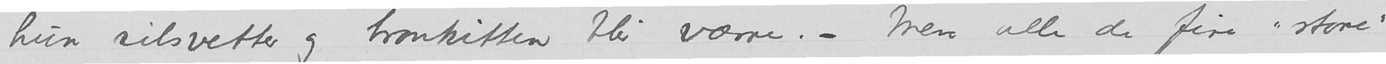hun silsvetter og bronkitten blir værre. – Men alle de fire «store»
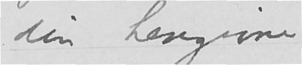din hengivne
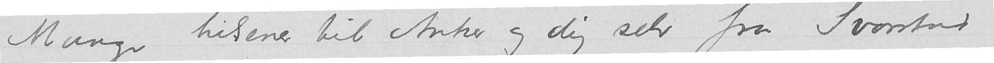Mange hilsener til Anker og dig selv fra Svarstad og
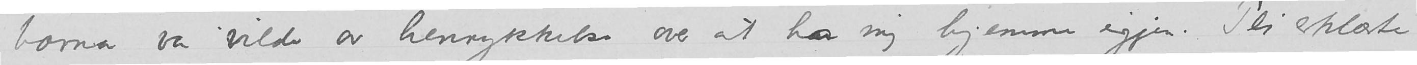barna var vilde av henrykkelse over at ha mig hjemme igjen. Pei erklærte
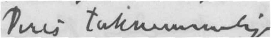Deres taknemmelige
